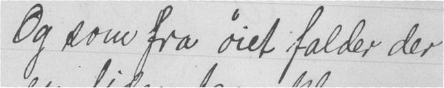Og som fra øiet falder der
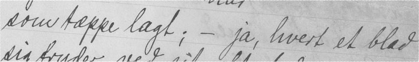som tæppe lagt; - ja, hvert et blad
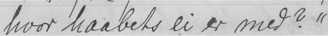hvor haabets ei er med?"
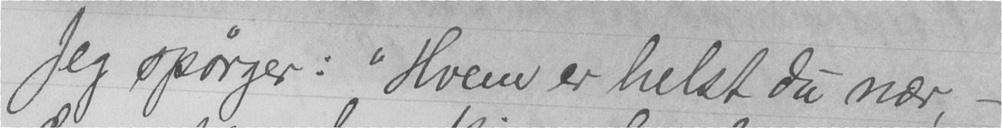Jeg spørger: "Hvem er helst du nær, -
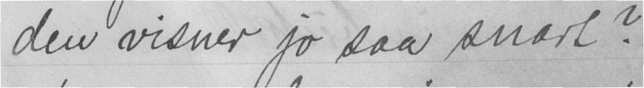den visner jo saa snart?
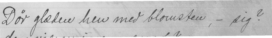Dør glæden hen med blomsten, - sig?
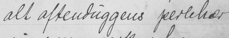alt aftenduggens perlehær
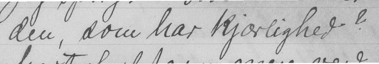den, som har kjærlighed?
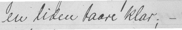en liden taare klar; -
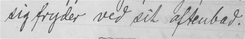sig fryder ved sit aftenbad.
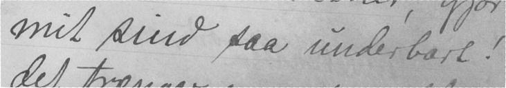mit sind saa underbart!
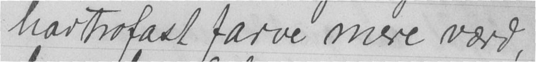har trofast farve mere værd,
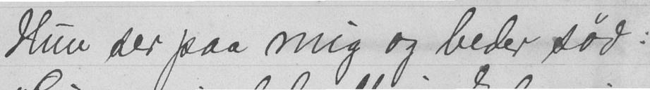Hun ser paa mig og beder sød:
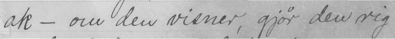ak - om den visner, gjør den rig
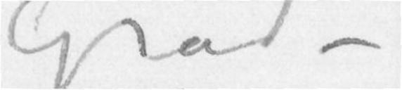Grad –
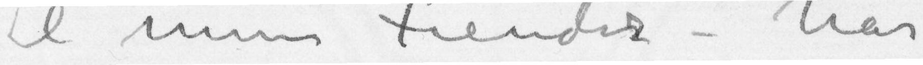til mine Fiender – har
